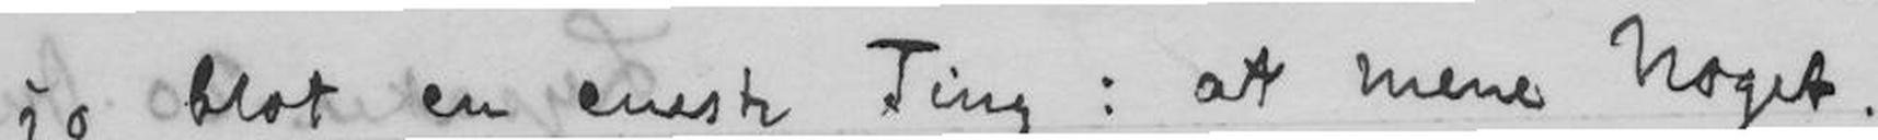jo blot en eneste Ting: at mene Noget.
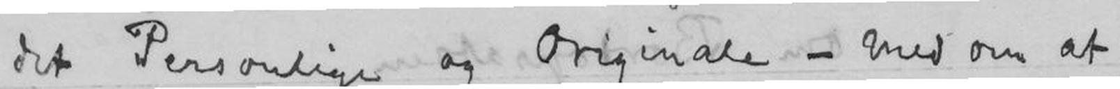det Personlige og Originale - med om at
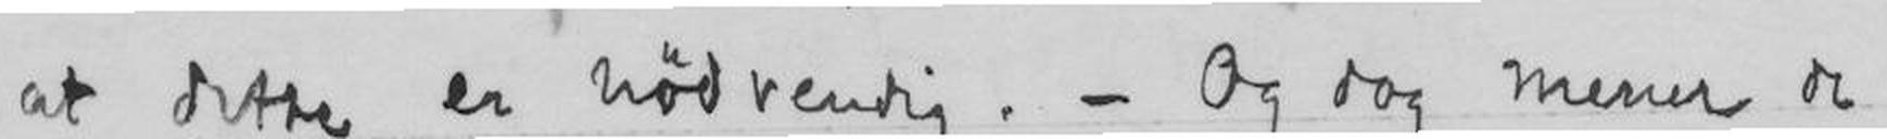at dette er nødvendig. - Og dog mener de
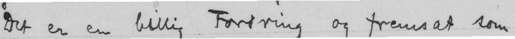Det er en billig Fordring og fremsat som
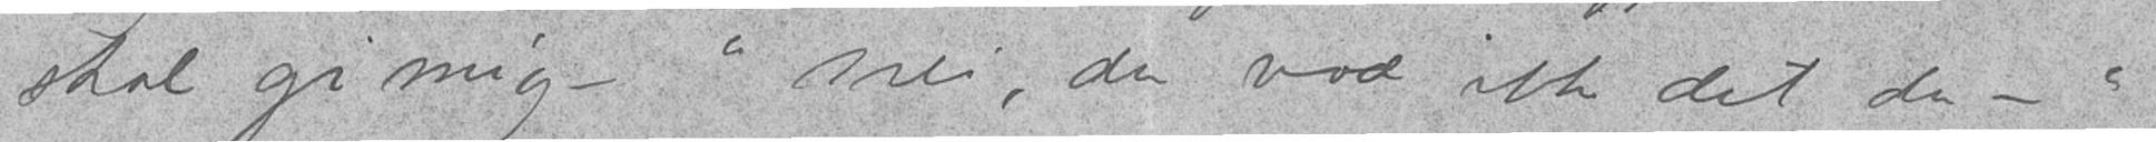skal gi meg —" "Nei, du ved ikke det du —"
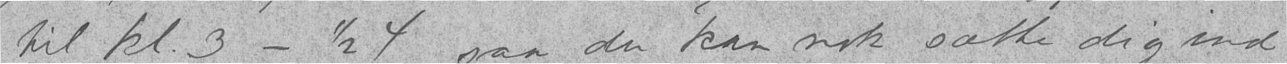til kl. 3 - ½4, saa du kan nok sætte dig ind
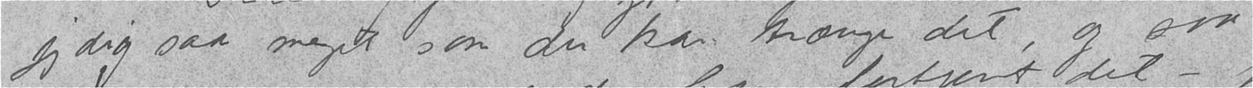jeg dig saa meget som du kan trænge det, og saa
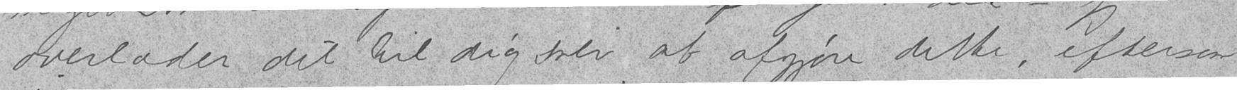overlader det til dig selv at afgjøre dette, eftersom
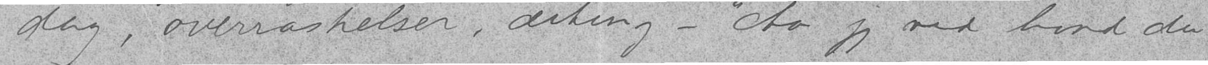dag, overraskelser, ærting — "Aa jeg ved hva du
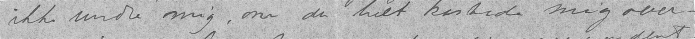ikke undre mig, om du helt kastede mig over-
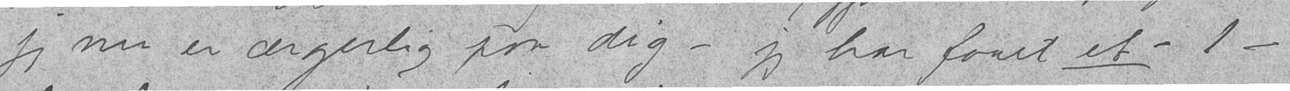jeg nu er ærgerlig paa dig — jeg har faaet et — 1 —
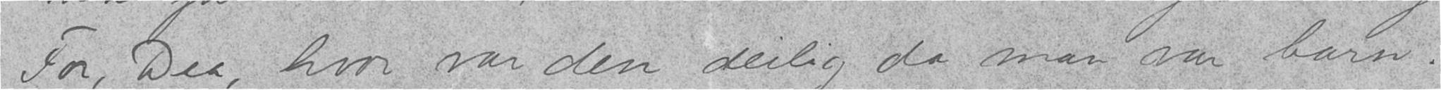For, Dea, hvor var den deilig da man var barn!
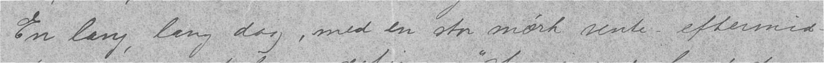En lang, lang dag, med en stor mørk vente-eftermid-
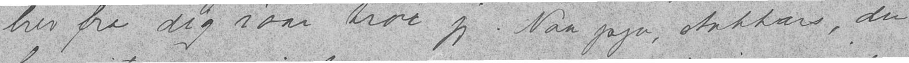brev fra dig iaar tror jeg. Naa jaja, stakkars, du
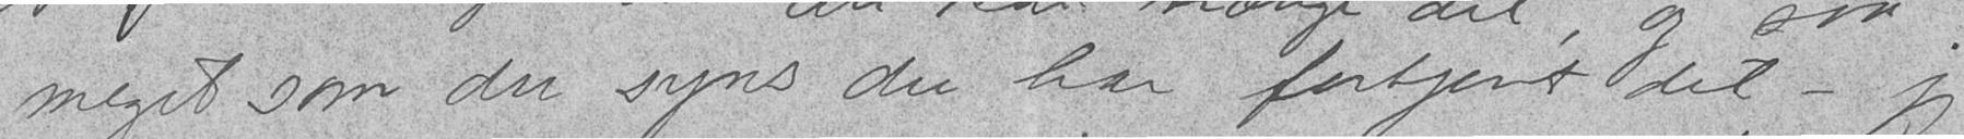meget som du syns du har fortjent det — jeg
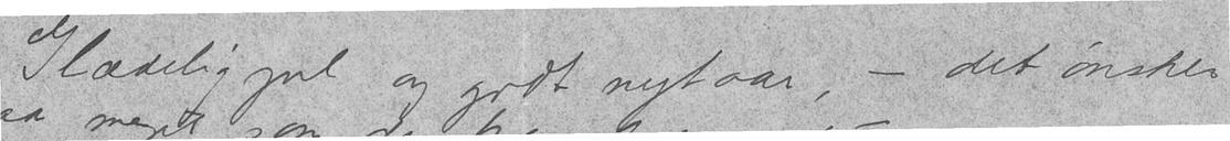Glædelig jul og godt nytaar, — det ønsker
